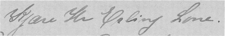Kjære Hr Erling Lone.
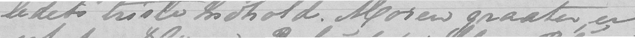ledets triste Indhold. Moren graater, er
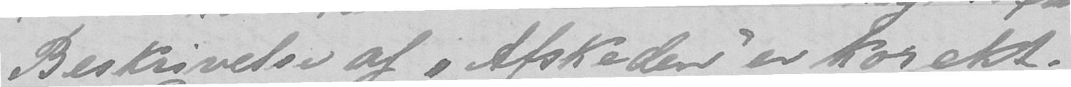Beskrivelse af "Afskeden" er korekt.
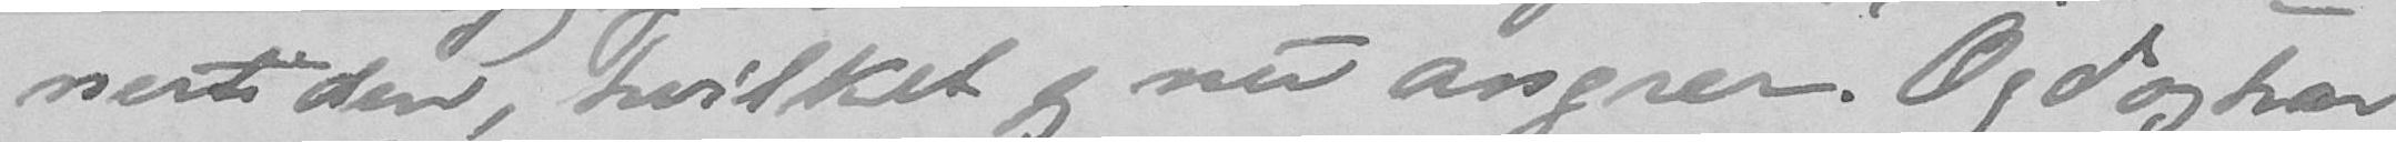nertiden, hvilket jeg nu angrer. Og dog har
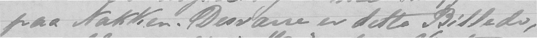paa Nakken. Desværre er dette Billede,
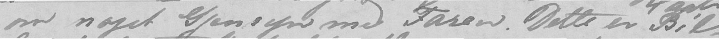om noget Gjensyn med Faren. Dette er Bil-
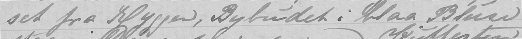set fra Ryggen, Bybudet i blaa Bluse
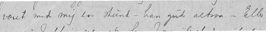været med mig en stund – han gud altsaa. - Eller
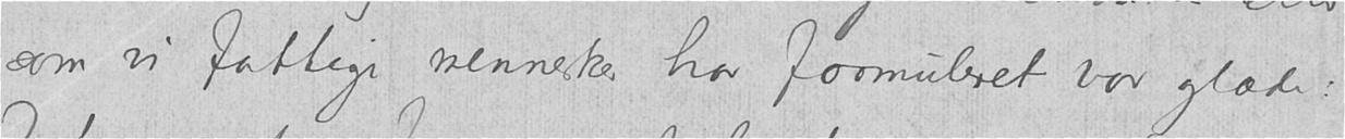som vi fattige mennesker har formuleret vor glæde:
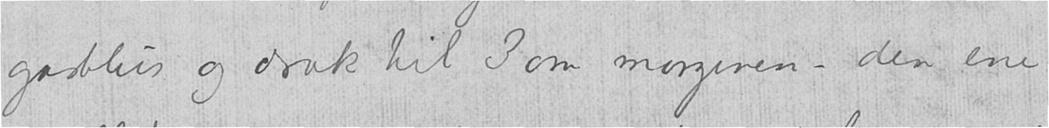gasblus og drak til 3 om morgenen – den ene
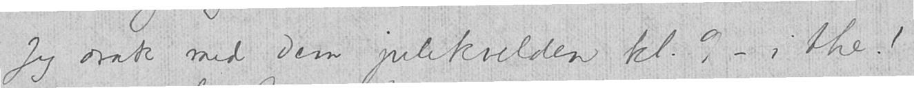Jeg drak med Dem julekvelden kl. 9 – i the!
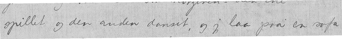spillet og den anden danset, og jeg laa paa en sofa
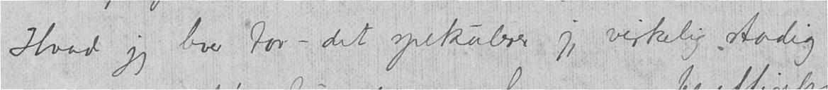Hvad jeg lever for - det spekulerer jeg virkelig stadig
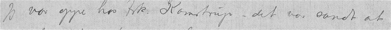Jeg var oppe hos Frk. Kamstrup – det var sandt at
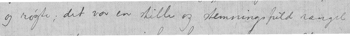og røgte; det var en stille og stemningsfuld rangel
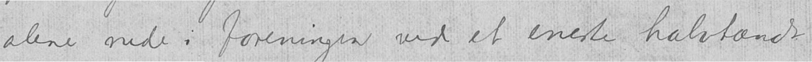alene nede i foreningen ved et eneste halvtændt
